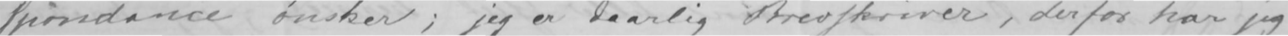spondance ønsker; jeg er daarlig Brevskriver, derfor har jeg
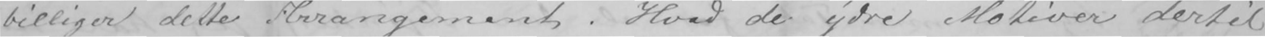billiger dette Arrangement. Hvad de ydre Motiver dertil
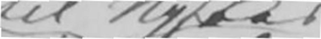til Nytaar
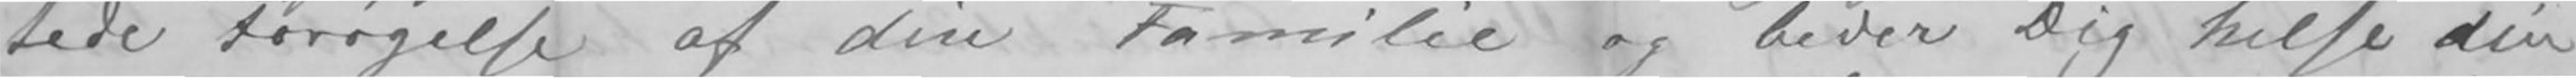tede Forøgelse af din Familie og beder Dig hilse din
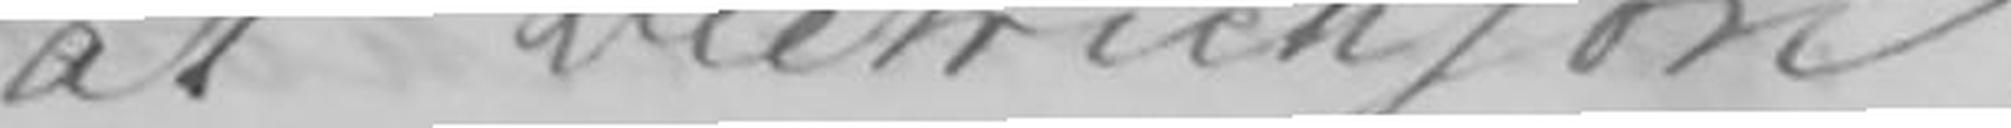at Dietrichson
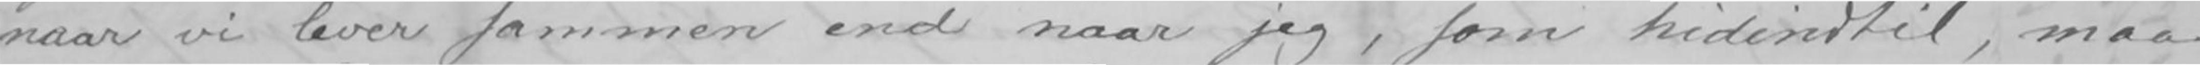naar vi lever sammen end naar jeg, som hidindtil, maa
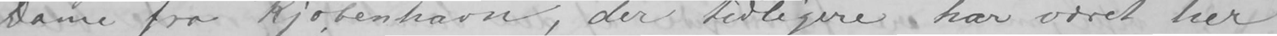Dame fra Kjøbenhavn, der tidligere har været her
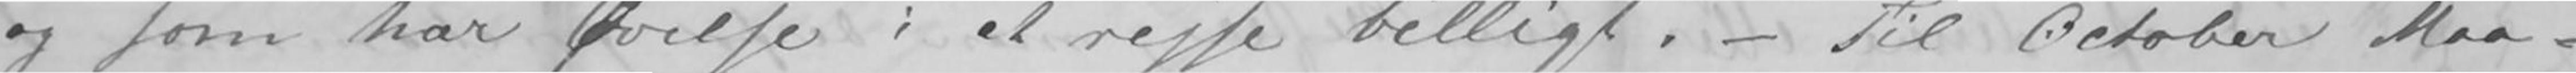og som har Øvelse i at rejse billigt.- Til October Maa-
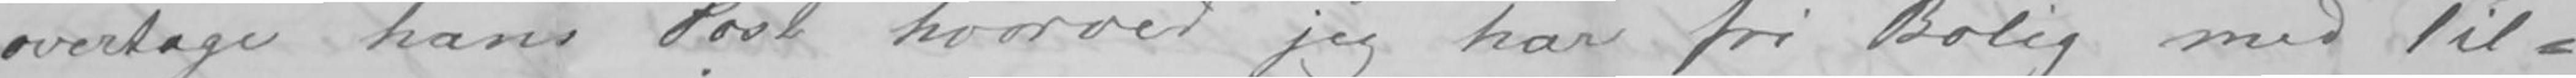overtage hans Post hvorved jeg har fri Bolig med Til-
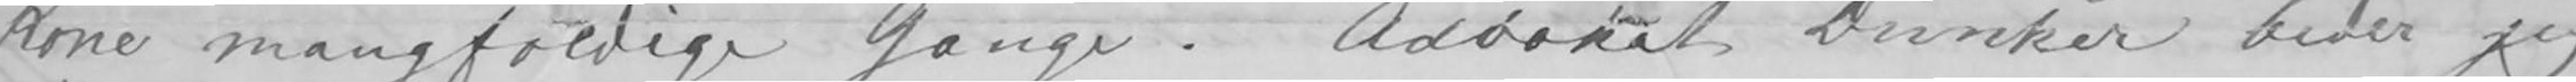Kone mangfoldige Gange. Advokat Dunker beder jeg
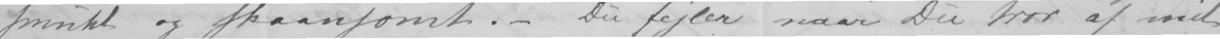smukt og skaansomt.- Du fejler naar Du tror af mit
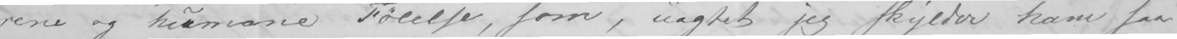rene og humane Følelse, som, uagtet jeg skylder ham saa
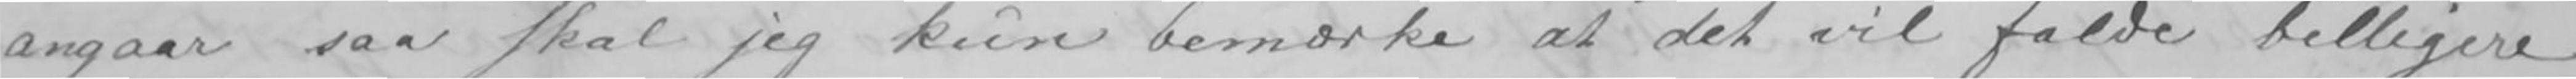angaar saa skal jeg kun bemærke at det vil falde billigere
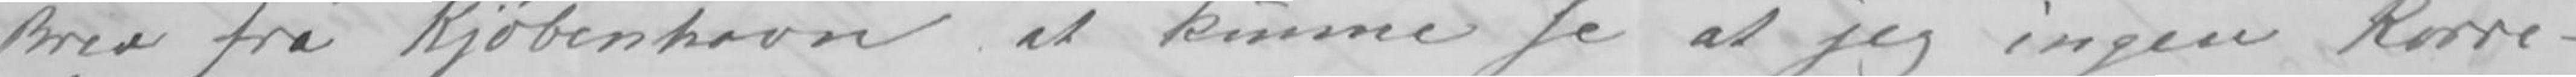Brev fra Kjøbenhavn at kunne se at jeg ingen Korre-
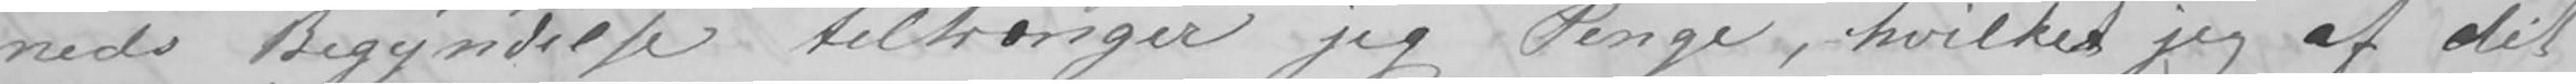neds Begyndelse tiltrænger jeg Penge, hvilket jeg af dit
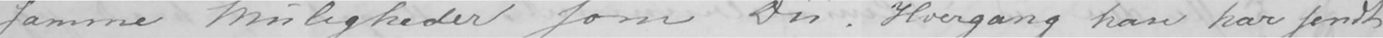samme Muligheder som Du. Hvergang han har sendt
